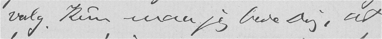valg. Kun maa jeg bede Dig, at
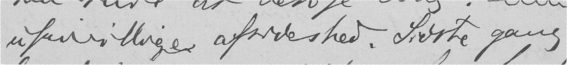ufrivillige afsideshed. Sidste gang
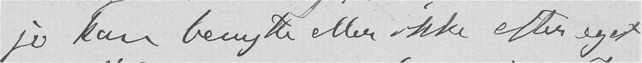jo kan benytte eller ikke efter eget
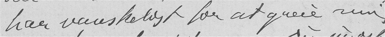har vanskeligt for at greie mig
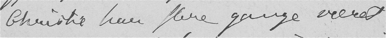Christie har flere gange været
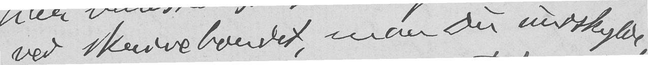ved skrivebordet, maa Du undskylde,
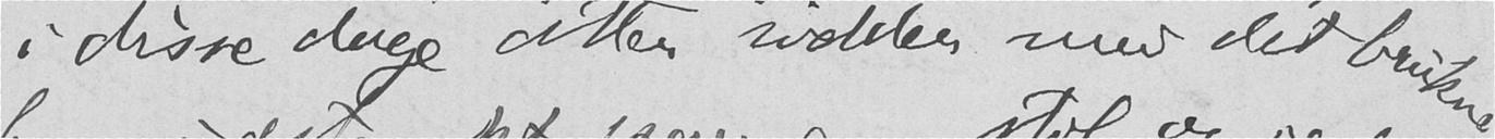i disse dage atter sidder med det brukne
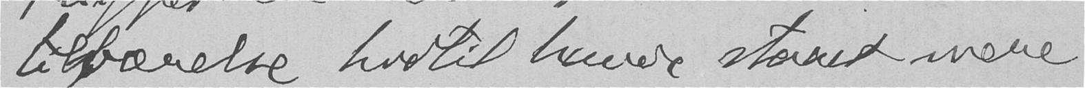tilværelse hidtil havde staaet mere
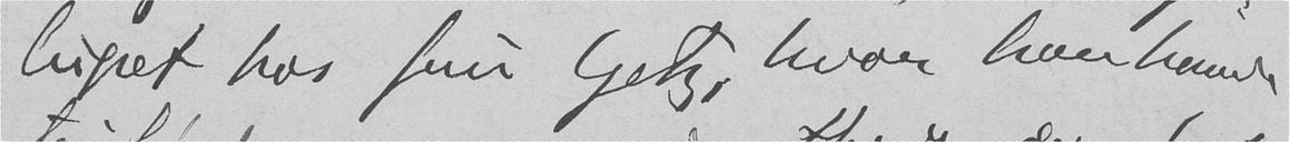lupet hos fru Getz, hvor han havde
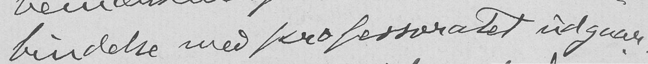bindelse med professoratet udgaar.
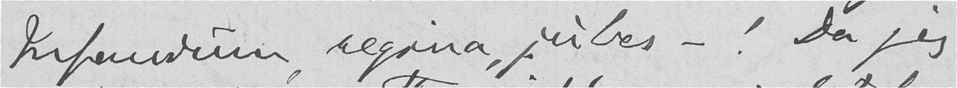Infandum, regina, jubes - ! Da jeg
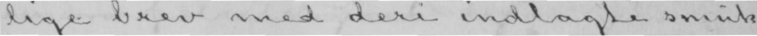lige brev med deri indlagte smuk-
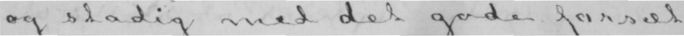og stadig med det gode forsæt
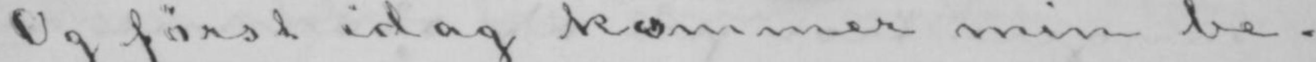Og først idag kommer min be-
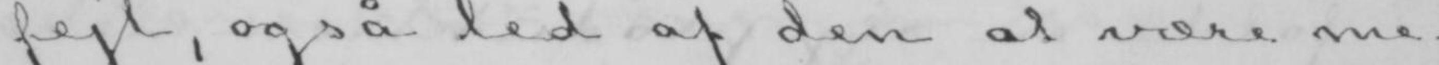fejl, også led af den at være me-
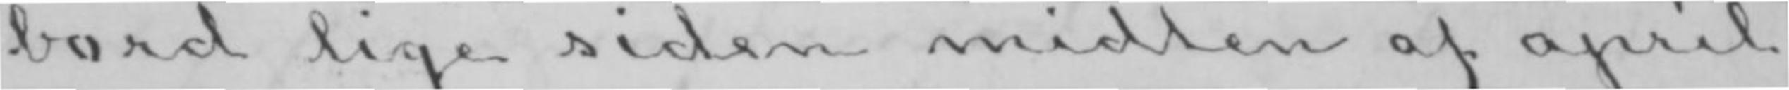bord lige siden midten af april,
